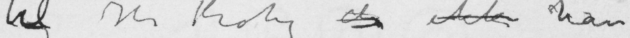til Hr Krohgikke han
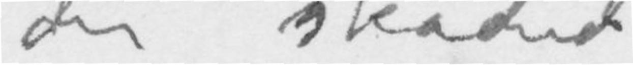der skadede
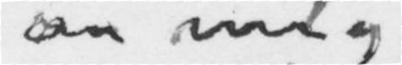om mig
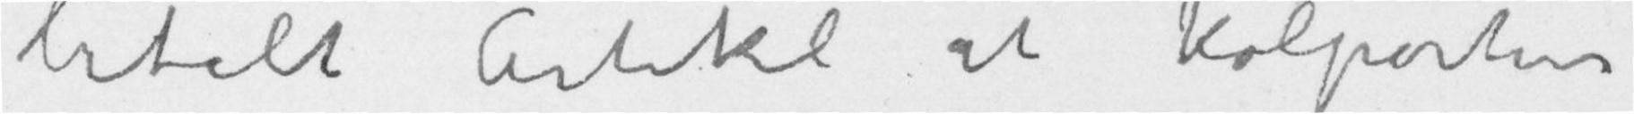betalt Artikel at kolportere
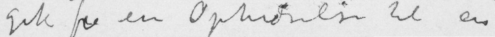gik fra en Ophidselse til en
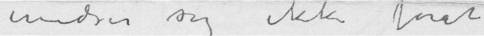undså sig ikke forat
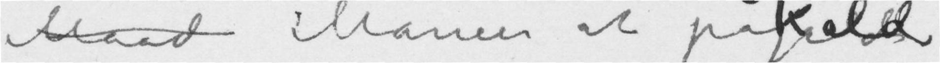Maade Maner at påkalde
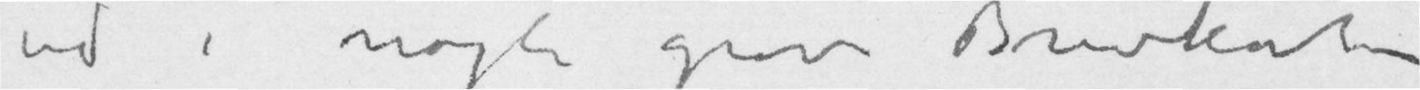ud i nogle grove Brevkort
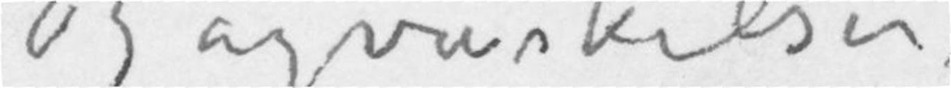Bagvaskelser
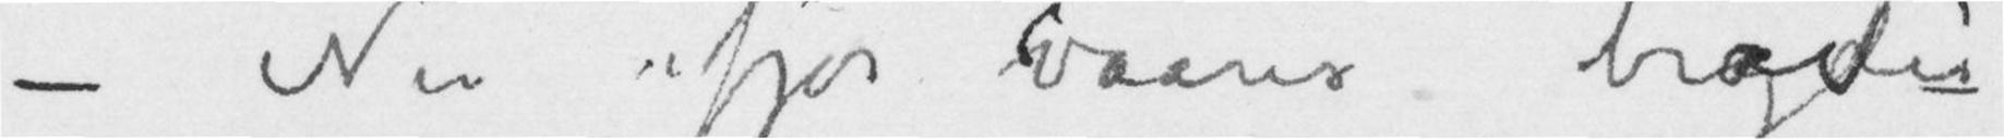– Nu ifjor vaares brød
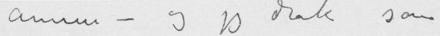annen – og jeg drak som
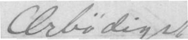Ærbødigst
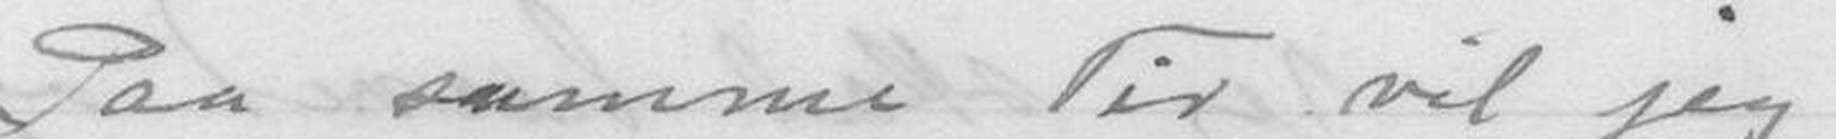Paa samme Tid vil jeg
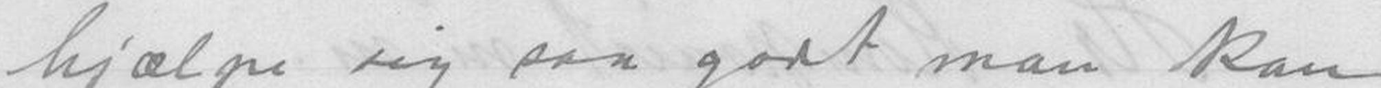hjælpe sig saa godt man kan
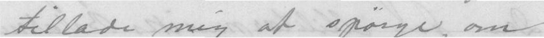tillade mig at spørge, om
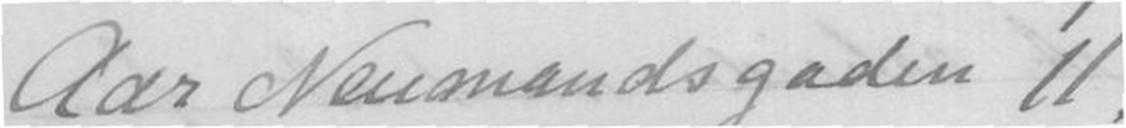Aar Neumandsgaden 11.
